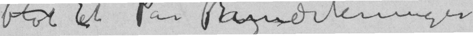blot Et par Bemerkninger
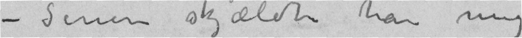– Senere skjældte han mig
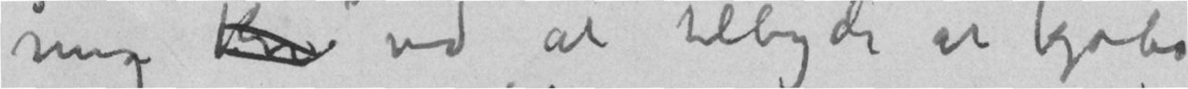migved at tilbyde at kjøbe
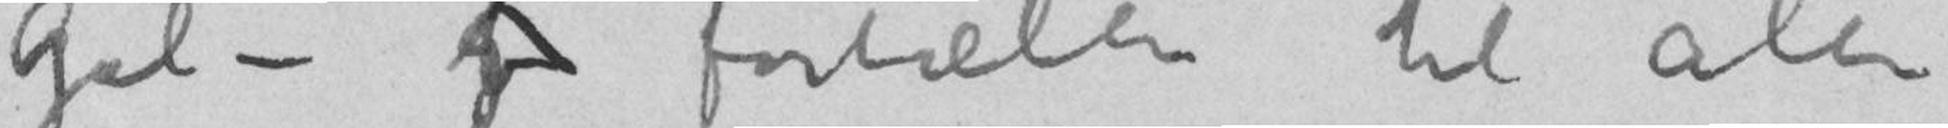Gal –fortælle til alle
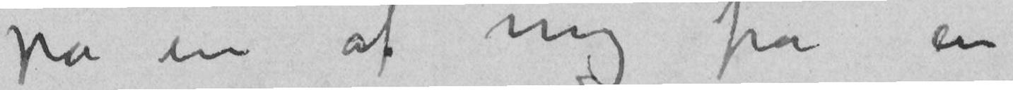på en af mig fra en
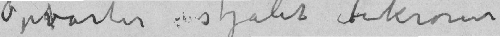Opvarter stjålet Tikroner
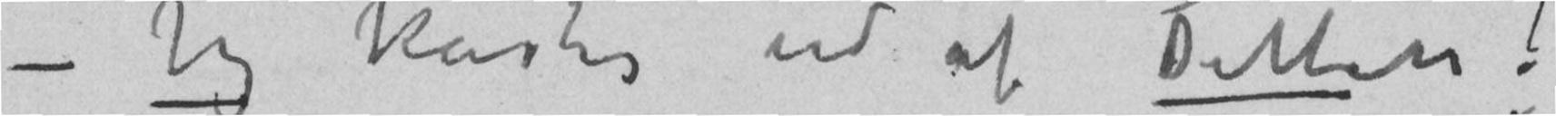– Jeg kastes ud av Ditten!
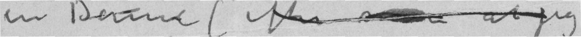en Dame (efterat jeg
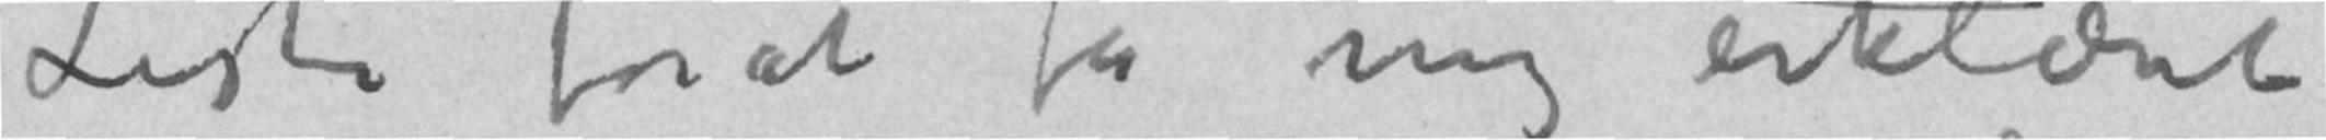Liste forat få mig erklæret
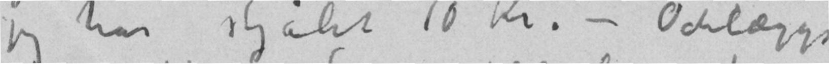jeg har stjålet 10 Kr. – Ødelegge
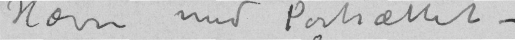Hævn med Portrættet –
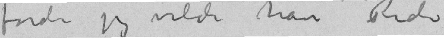fordi jeg vilde have Rede
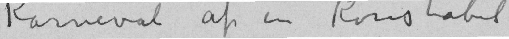Karneval af en Konstabel
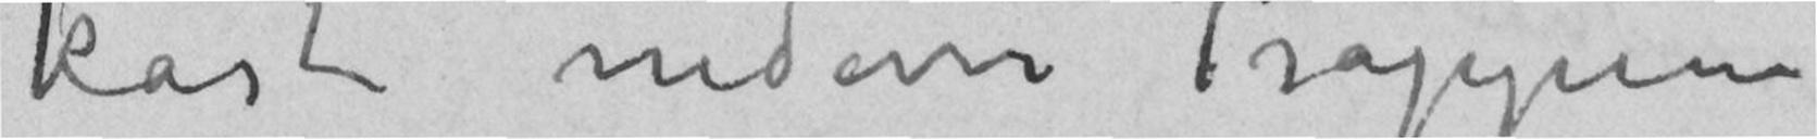kaste nedover Trappene
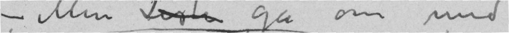Men Liste gå om med
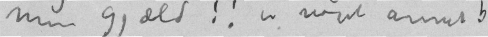min Gjæld!! er noget annet!
